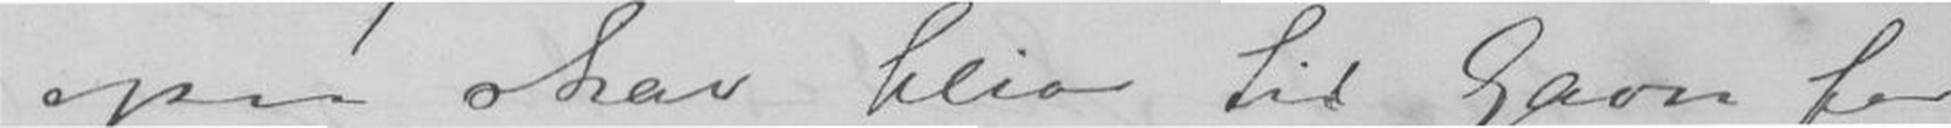ogsaa skal blive til Gavn for
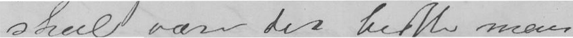skal være det bedste man
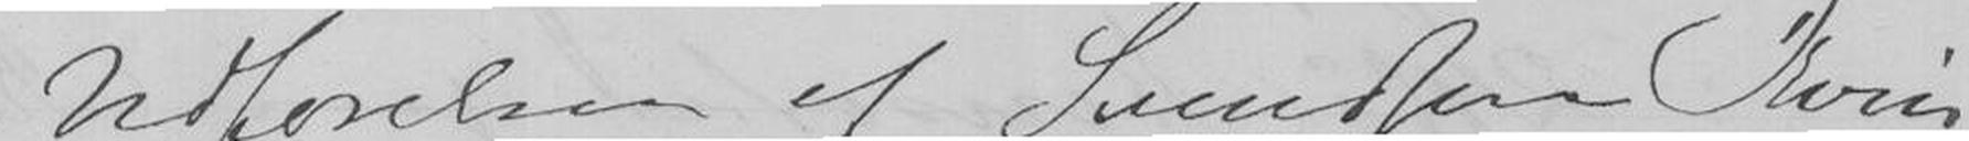Udførelsen af Svendsen Kvin
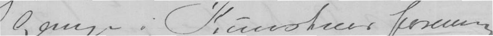Gange i Kunstner foreningen
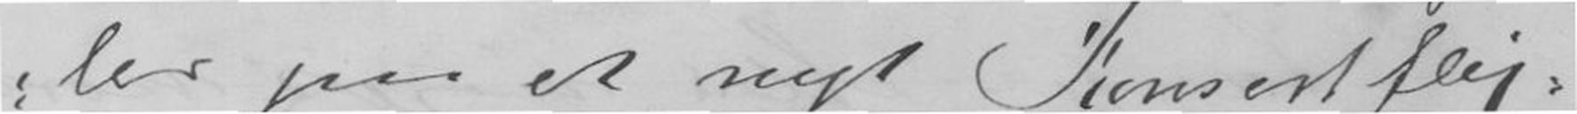ler paa et nyt Konsertfly-
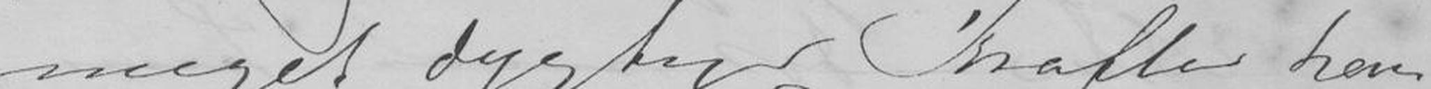meget dygtige Kræfter han
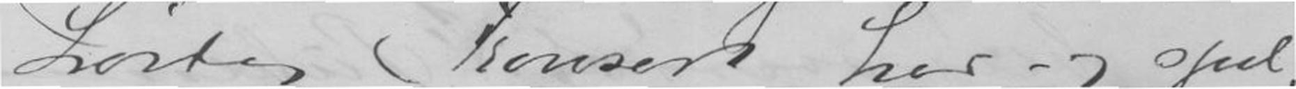Lørdag Konsert her og spil-
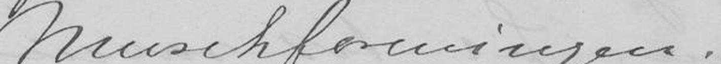Musikforeningen. -
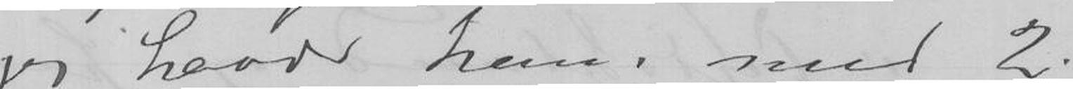jeg havde ham, med 2.
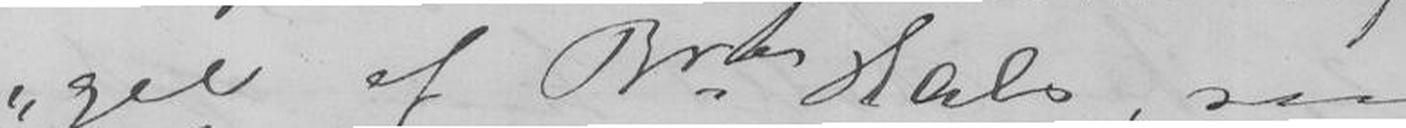gel af Brdr Hals, som
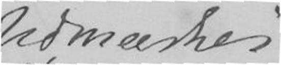Udmærket
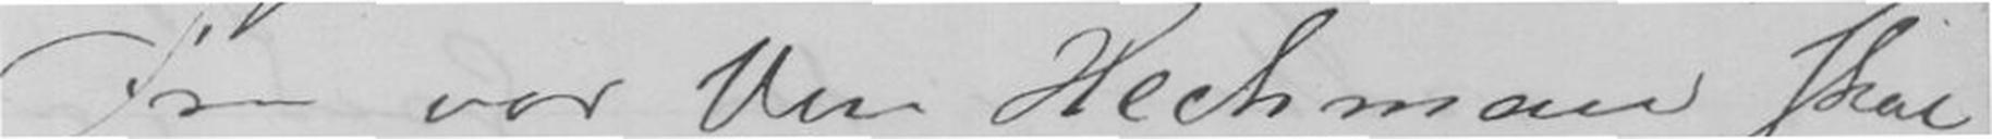Fra vor Ven Hechman skal
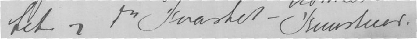tet og Din Kvartet - Kunstner.
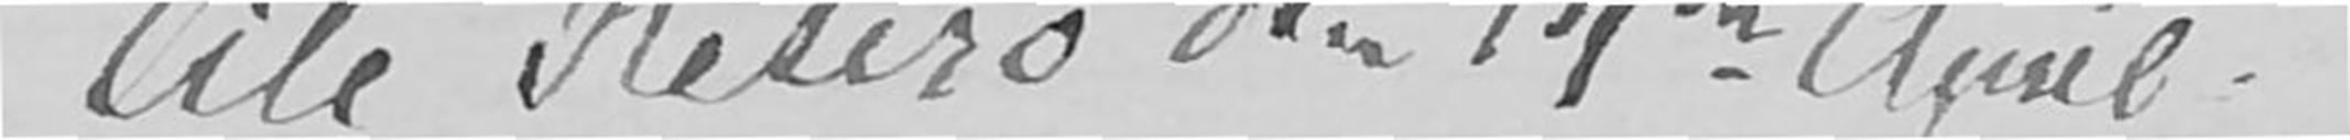Cité Retiro den 14de April.
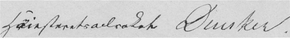Høiesteretsadvokat Dunker.
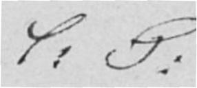S: T:
